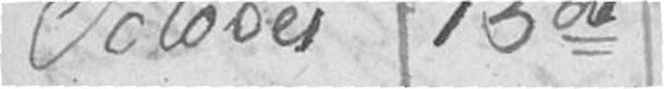October 13de
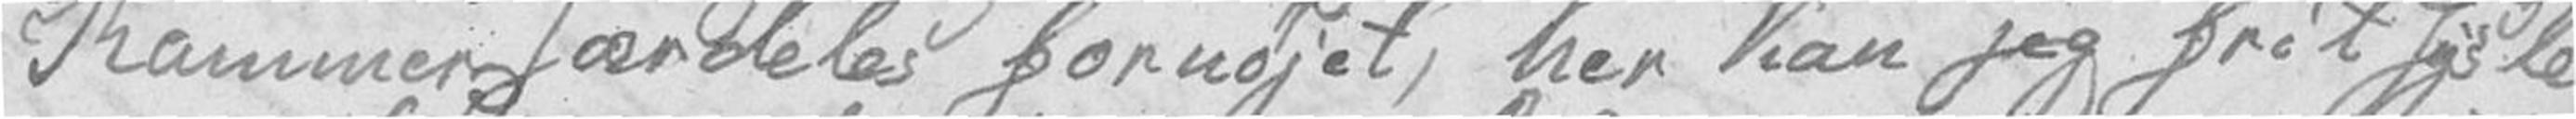Kammer særdeles fornøjet, her kan jeg frit sysle
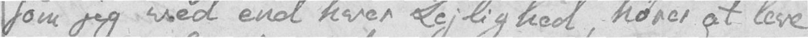som jeg ved end hver Lejlighed, hører at leve
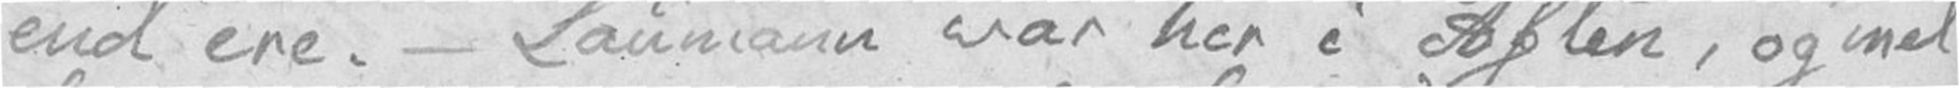end ere. – Laumann var her i Aften, og med
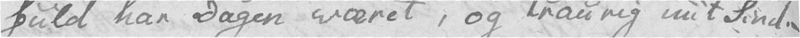fuld har Dagen været, og traurig mit Sind.
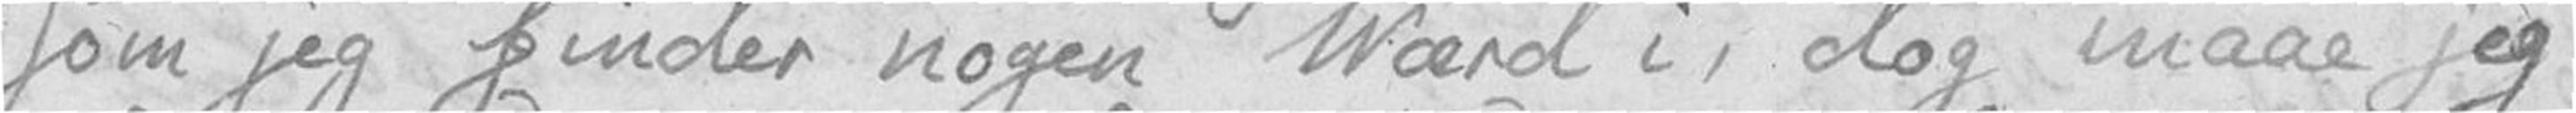som jeg finder nogen Værd i, dog maae jeg
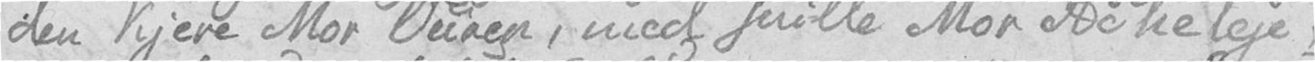den kjere Mor Ouren, med snille Mor Acheleje,
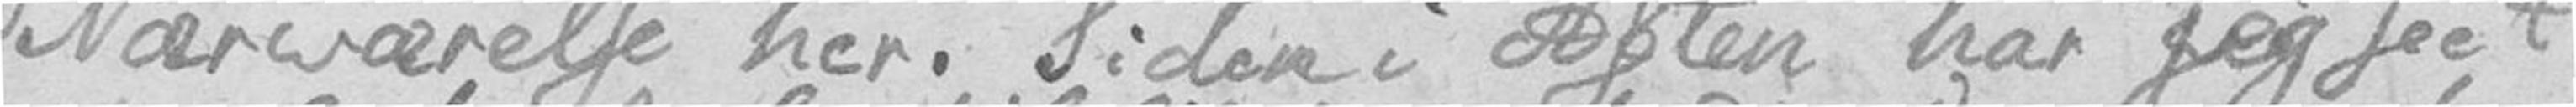Nærværelse her. Siden i Aften har jeg seet
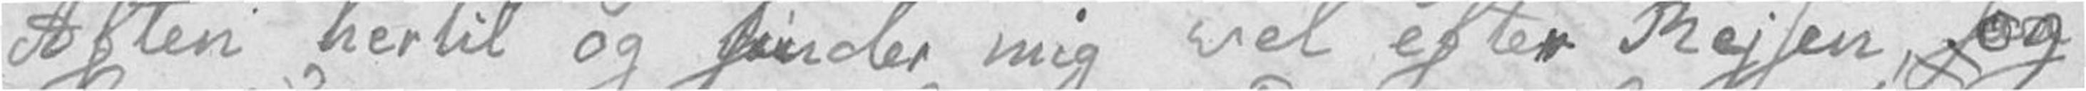Aften hertil og finder mig vel efter Rejsen, og
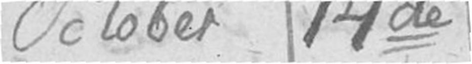October 14de
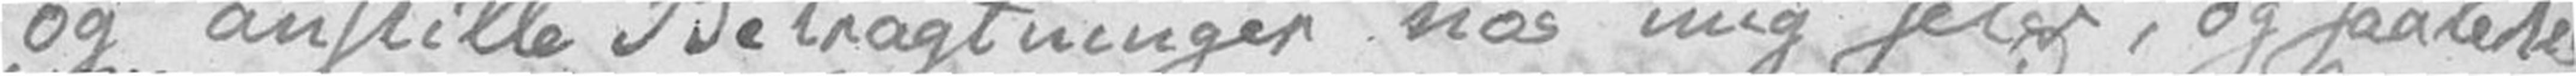og anstille Betragtninger hos mig selv, og saaledes
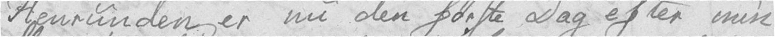Henrunden er nu den første Dag efter min
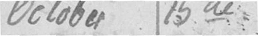October 15de
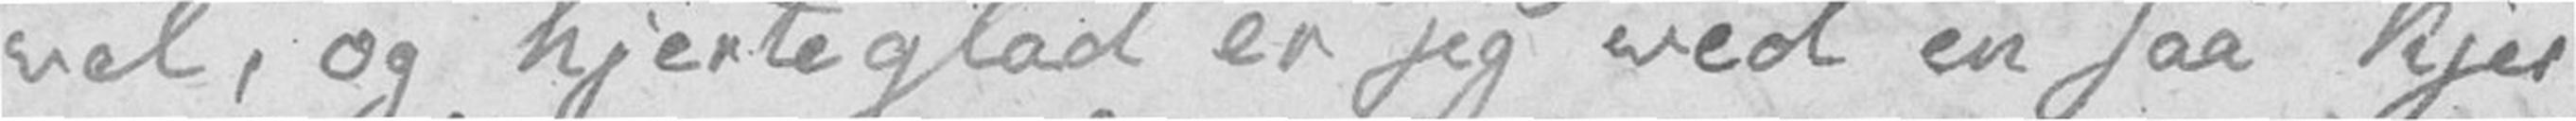vel, og hjerteglad er jeg ved en saa kjer
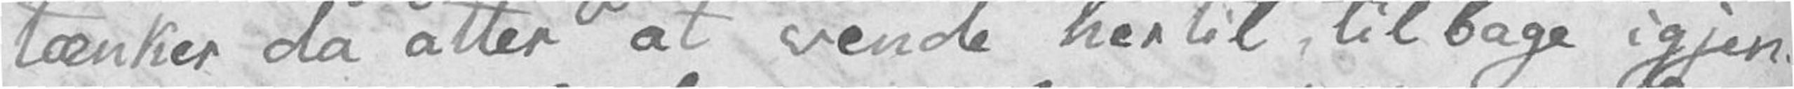tænker da atter at vende hertil, tilbage igjen
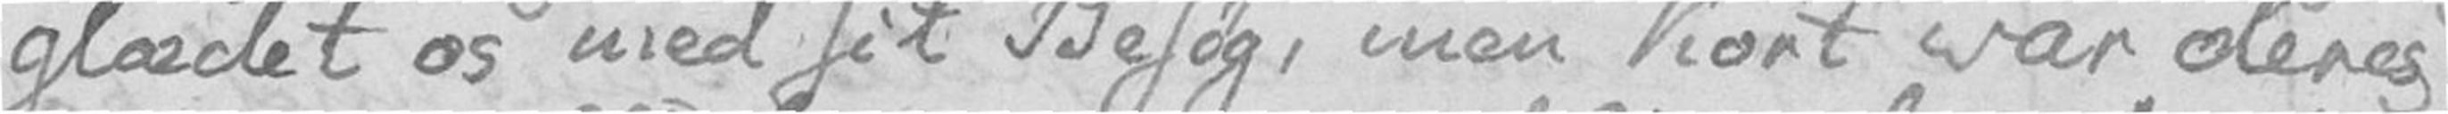glædet os med sit Besøg, men kort var deres
